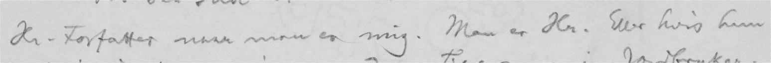Hr. Forfatter naar man er mig. Man er Hr. Eller hvis hun
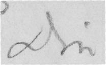Din
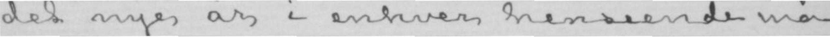det nye år i enhver henseende må
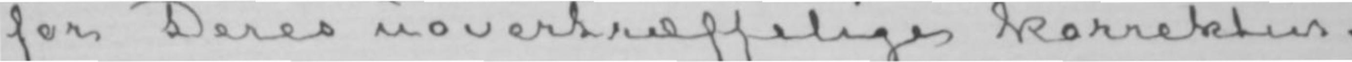for Deres uovertræffelige korrektur-
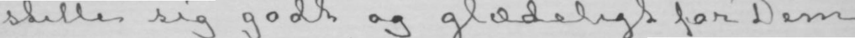stille sig godt og glædeligt for Dem
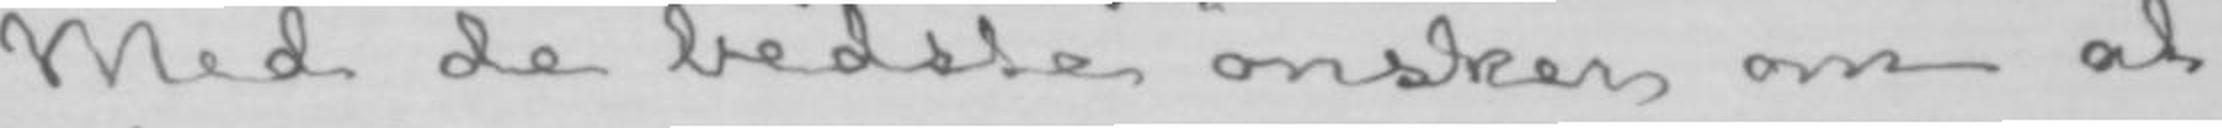Med de bedste ønsker om at
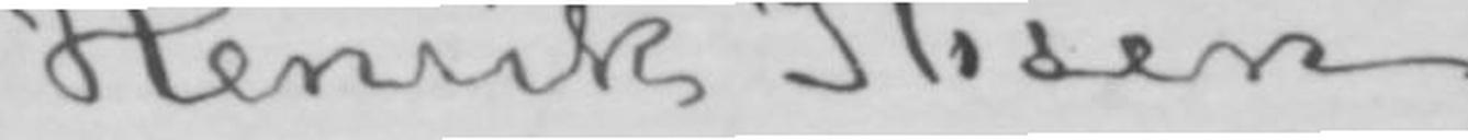Henrik Ibsen.
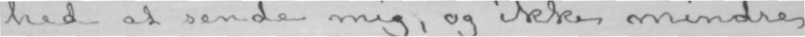hed at sende mig, og ikke mindre
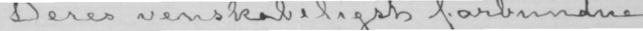Deres venskabeligst forbundne
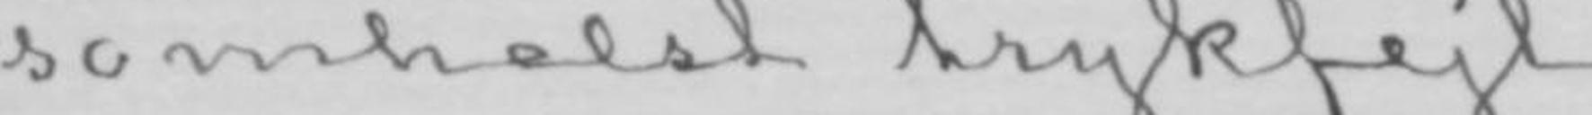somhelst trykfejl.
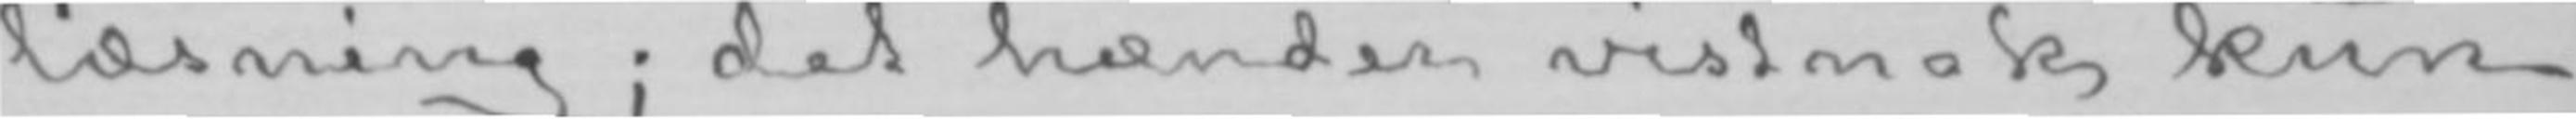læsning; det hænder vistnok kun
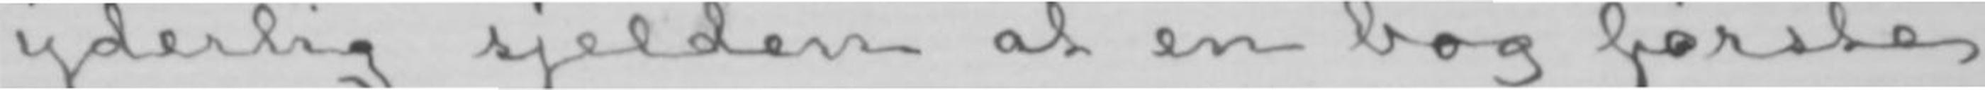yderlig sjelden at en bog første
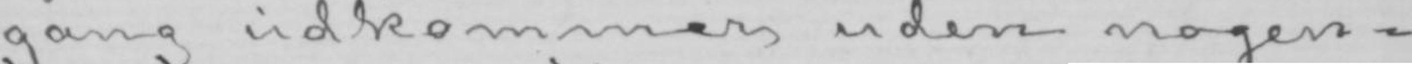gang udkommer uden nogen-
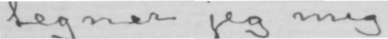tegner jeg mig
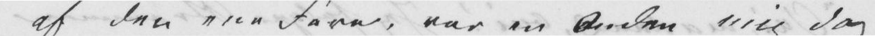af den ene Fare, var en Anden mig dog
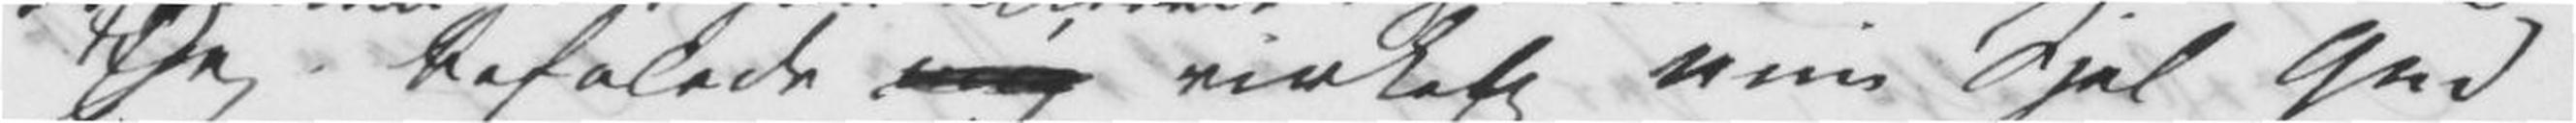Jeg befalede mig virkelig min Sjel Gud
